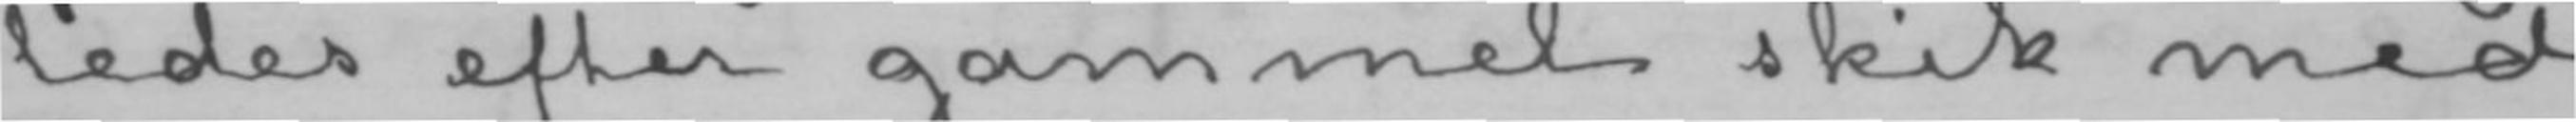ledes efter gammel skik med
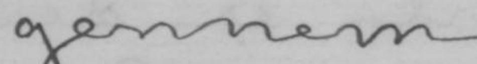gennem
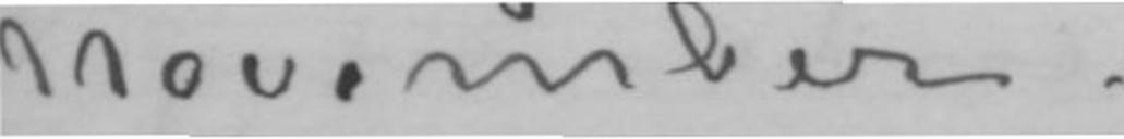November.
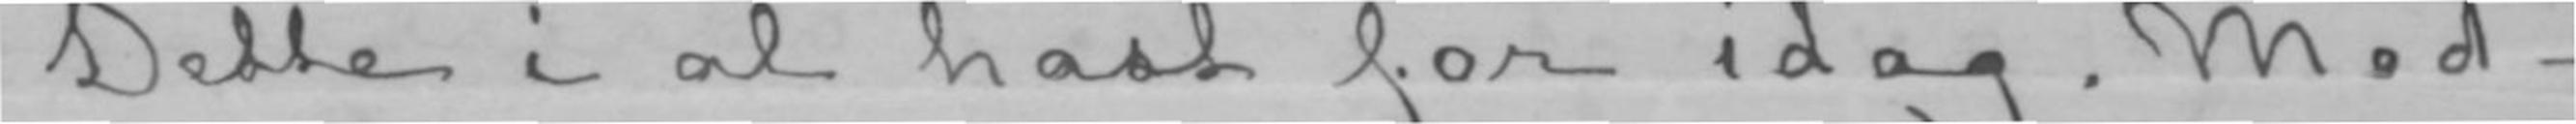Dette i al hast for idag. Mod-
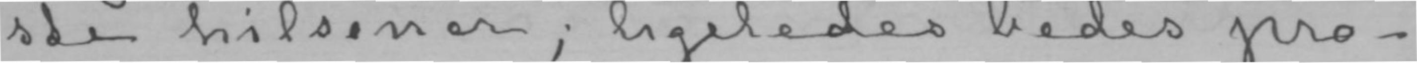ste hilsener; ligeledes bedes pro-
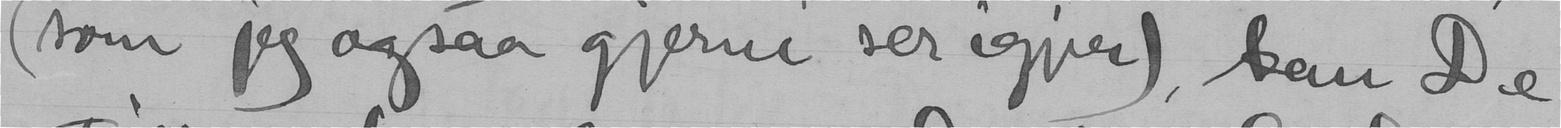(som jeg ogsaa gjerne ser igjen), kan De
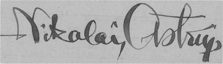Nikolai Astrup
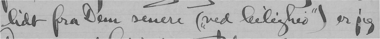lidt fra Dem senere ("ved leilighed") er jeg
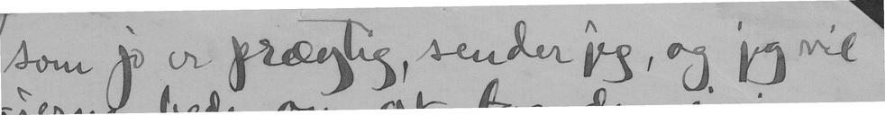som jo er prægtig, sender jeg, og jeg vil
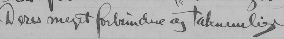Deres meget forbundne og taknemlige
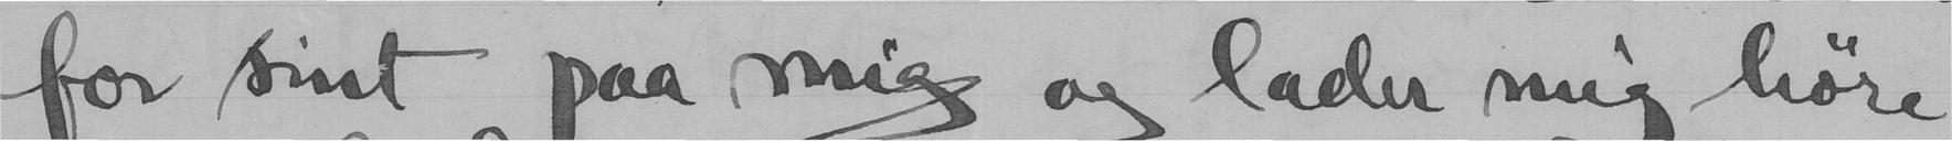for sint paa mig og lader mig høre
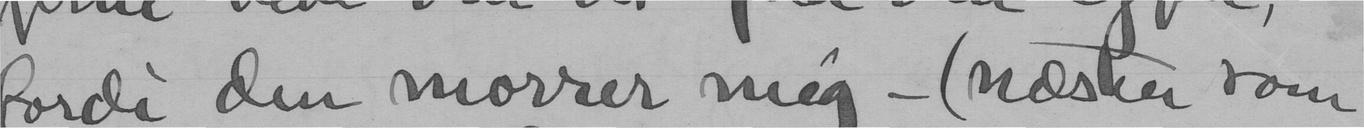fordi den morrer mig - (næsten som
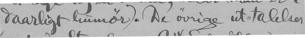daarligt humør). De øvrige ut-talelser
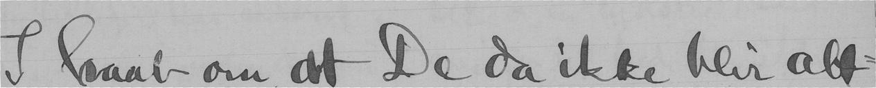I haab om at De da ikke blir alt-
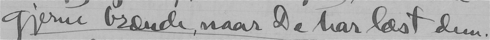gjerne brænde, naar De har læst dem.
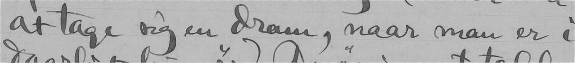at tage sig en dram, naar man er i
